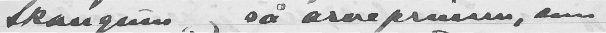Skaugum og så arveprinsen, som
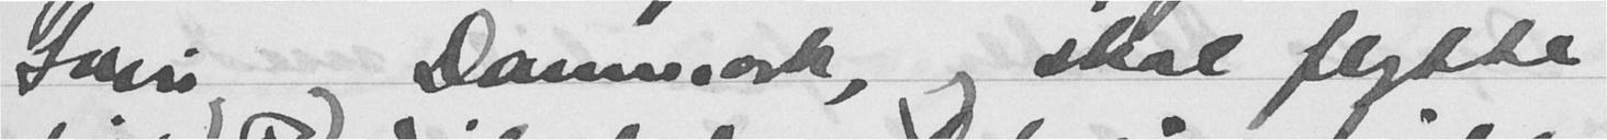Sverige og Dammark, og skal flytte
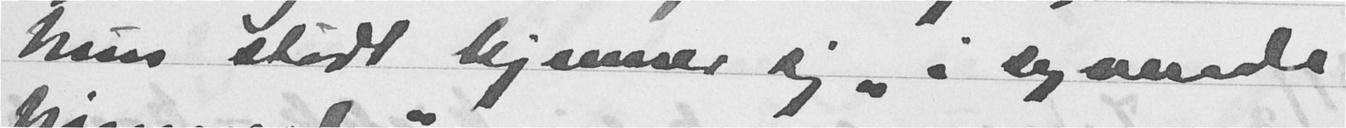hun stødt kjenner sig "i syvende
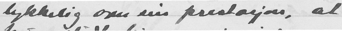lykkelig over sin prestasjon, at
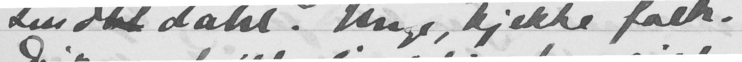Lind Dahl. Unge, kjekke folk.
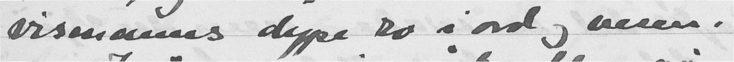vismanns dype ro i ord og vesen.
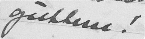guttene!
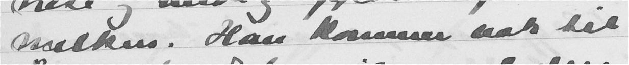melken. Han kommer nok til
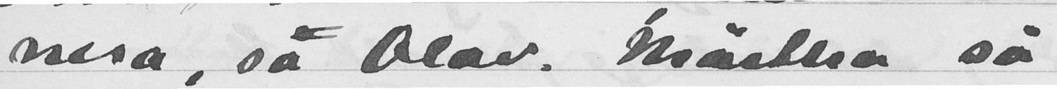nesa, sa Olav. Märtha så
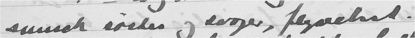svensk søster og svoger, fegvetst.
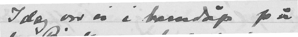I dag var vi i barndåp på
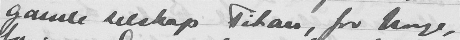gamle selskap Titan, for Norge,
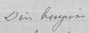Din hengivne
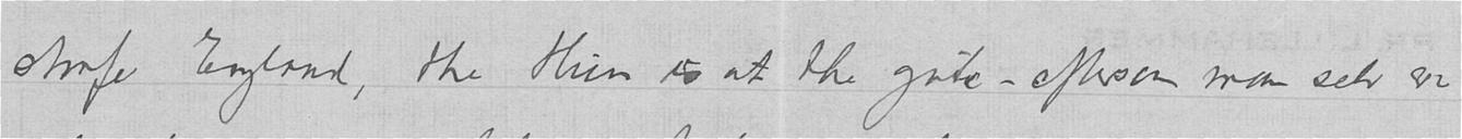strafe England, the Hun is at the gate – eftersom man selv er
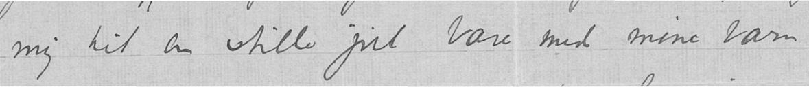mig til en stille jul bare med mine barn
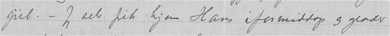jul. – Jeg selv fik hjem Hans iformiddags og glæder
